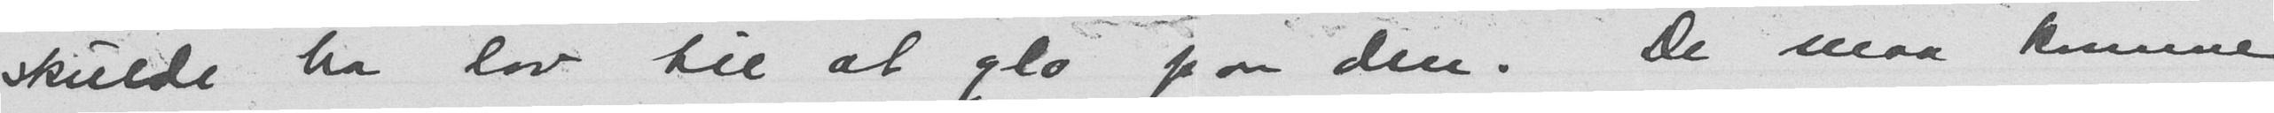skulde ha liv til at glo paa den. De maa komme
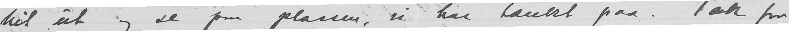hit ut og se paa plassen, vi har tænkt paa. Tak for
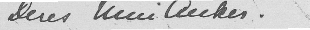Deres Nini Anker.
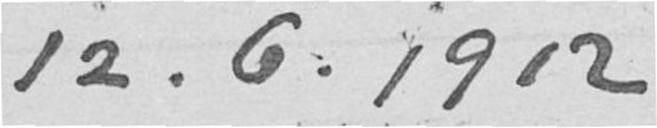12. 6. 1912
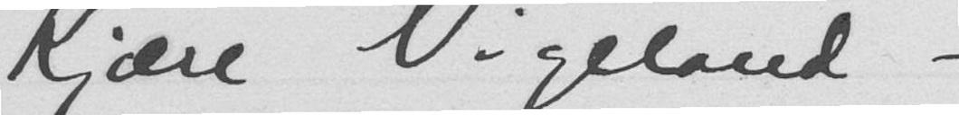Kjære Vigeland -
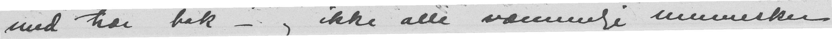med trær bak - og ikke alle væmmelige mennesker
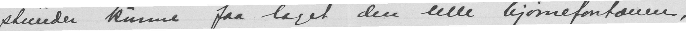stunder kunne faa laget den lille bjørnefontænen,
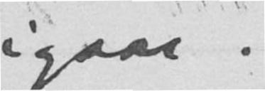igaar.
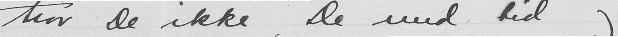Tror De ikke De med tid og
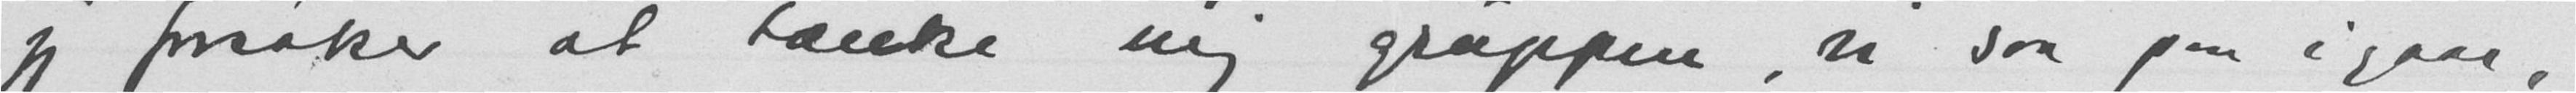jeg forsøker at tænke mig gruppen, vi saa paa igaar.
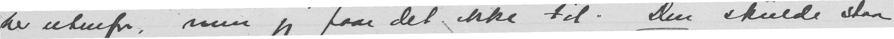her utenfor, men jeg faar det ikke til. Den skulde staa
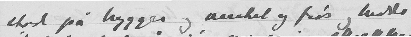stod på bryggen og ventet og frøs og hadde
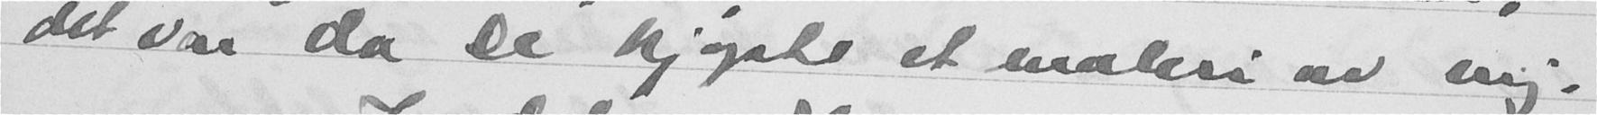det var da De kjøpte et maleri av mig.
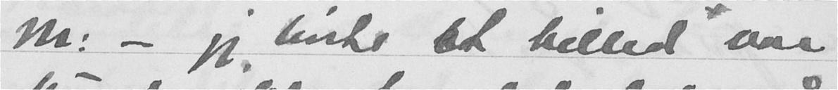M. - Jeg hørte et billed var
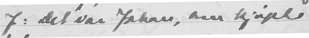J: det var Johan, han kjøpte
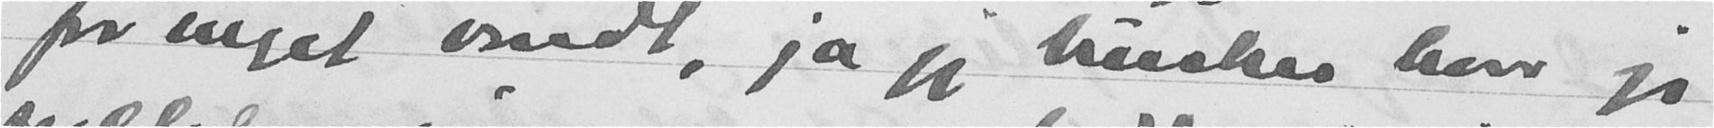for meget vondt, ja jeg husker hvor jeg
